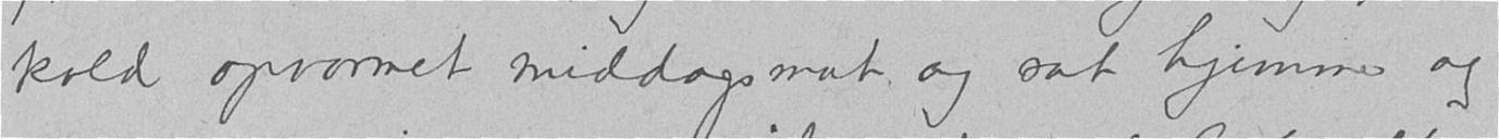kold opvarmet middagsmat og sat hjemme og
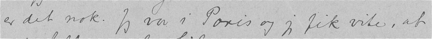er det nok. Jeg var i Paris og jeg fik vite, at
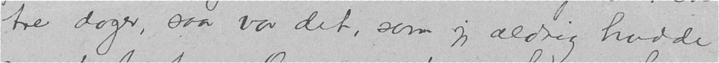tre dager, saa var det, som jeg aldrig hadde
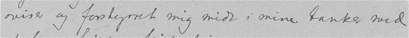aviser og forstyrret mig midt i mine tanker med
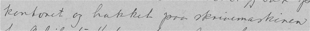kontoret og hakket paa skrivemaskinen
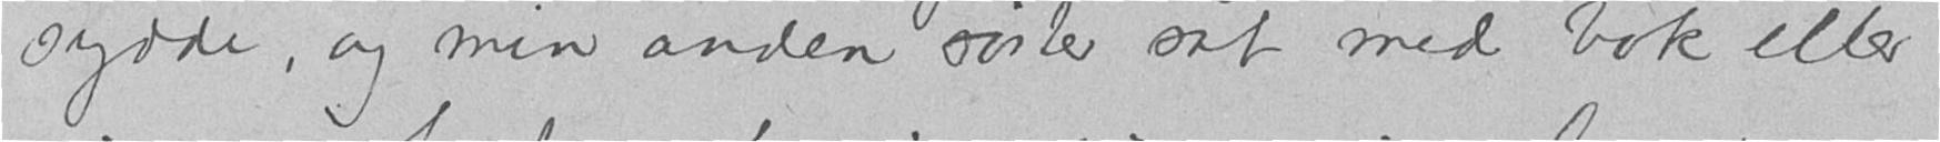sydde, og min anden søster sat med bok eller
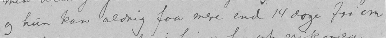og hun kan aldrig faa mere end 14 dage fri om
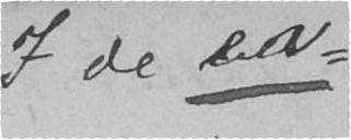I de eu
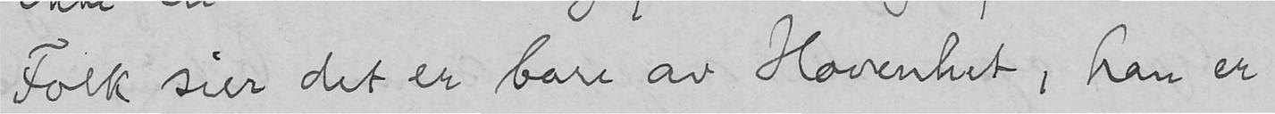Folk sier det er bare av Hovenhet, han er
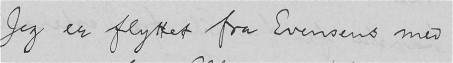Jeg er flyttet fra Evensens med
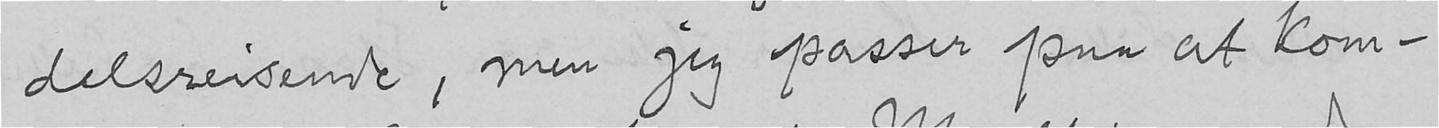delsreisende, men jeg passer paa at kom-
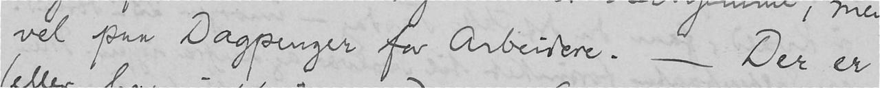vel paa Dagpenger for Arbeidere. - Der er
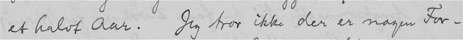et halvt Aar. Jeg tror ikke der er nogen For-
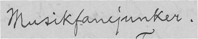Musikfanejunker.
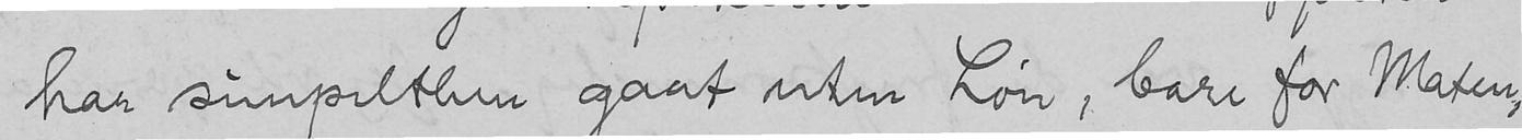har simpelthen gaat uten Løn, bare for Maten,
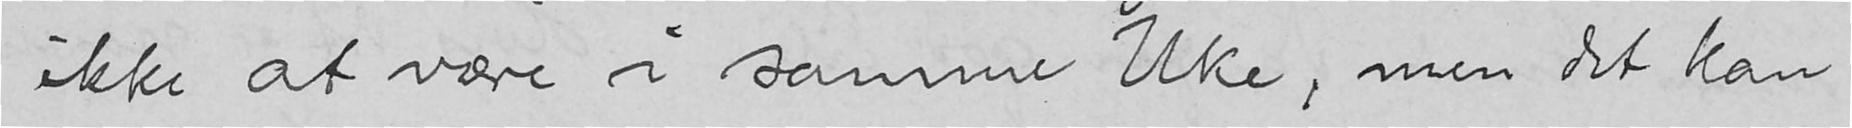ikke at være i samme Uke, men det kan
